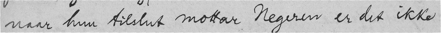naar hun tilslut mottar Negeren er det ikke
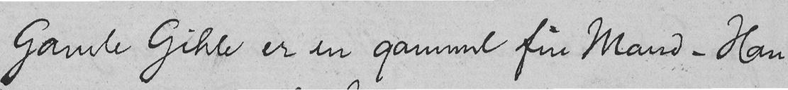Gamle Gihle er en gammel fin Mand. Han
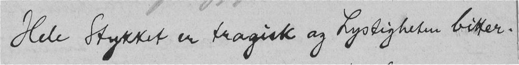Hele Stykket er tragisk og Lystigheten bitter.
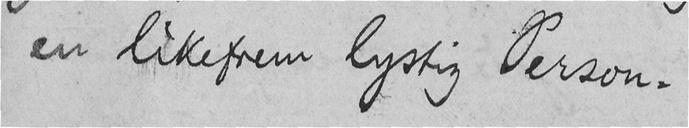en likefrem lystig Person.
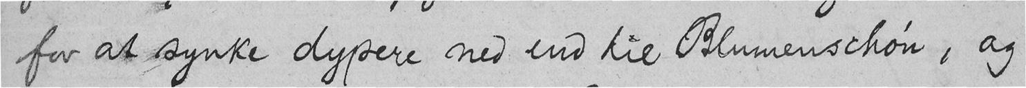for at synke dypere ned end til Blumenschøn, og
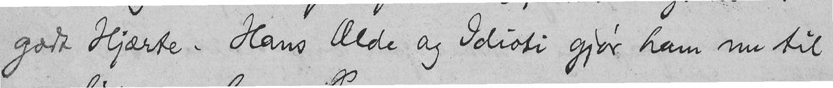godt Hjærte. Hans Ælde og Idioti gjør ham nu til
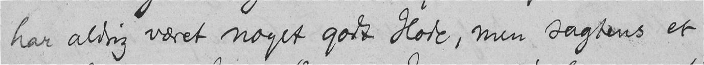har aldrig været noget godt Hode, men sagtens et
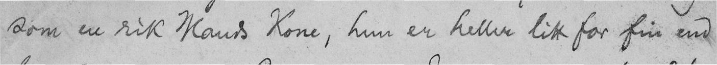som en rik Mands Kone, hun er heller litt for fin end
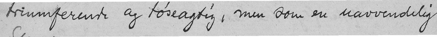triumferende og tøseagtig, men som en uavvendelig
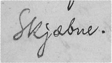Skjæbne.
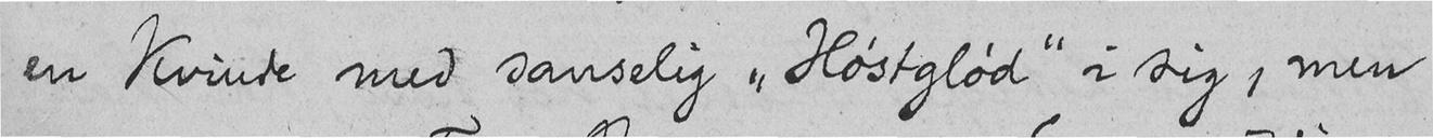en Kvinde med sanselig "Høstglød" i sig, men
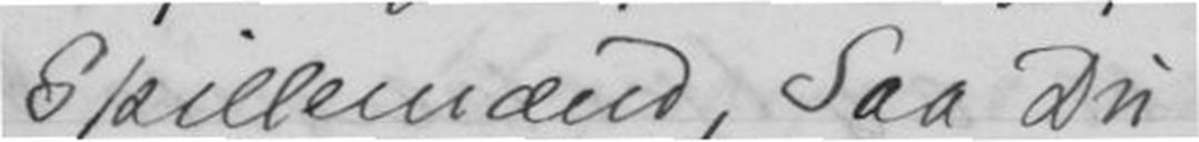Spillemænd, Saa Du
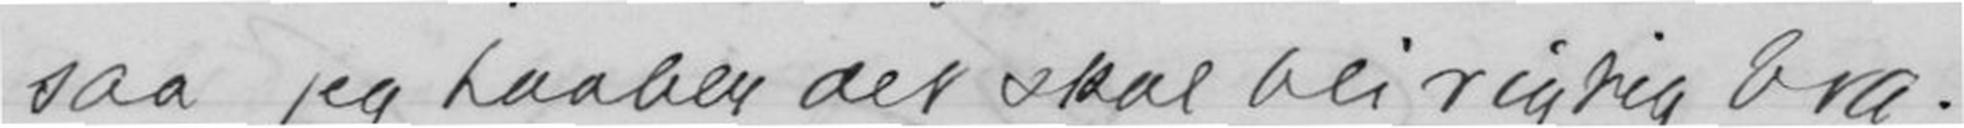saa jeg haaber det skal bli rigtig bra.
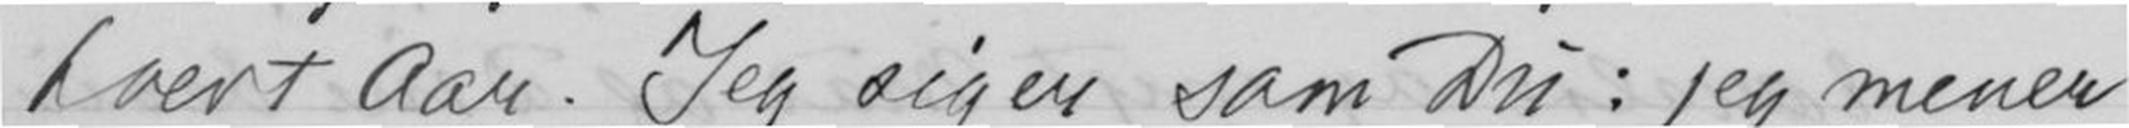hvert Aar. jeg siger som Du; jeg mener
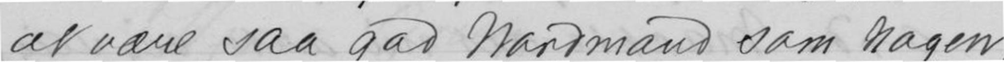at være saa god Nordmand som nogen,
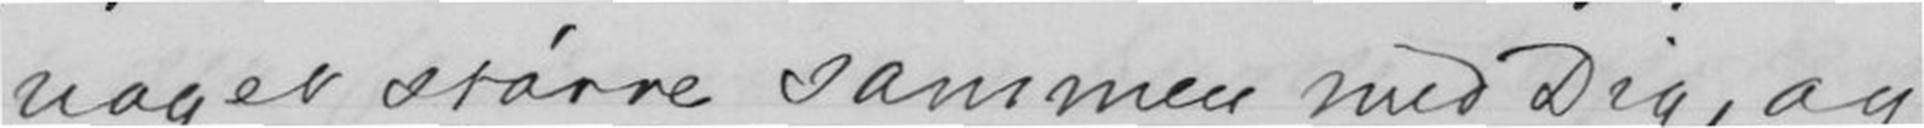noget større sammen med Dig, og
